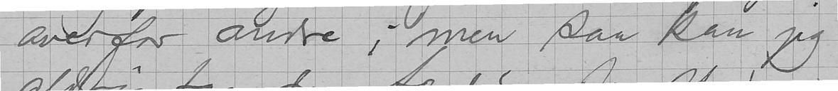overfor andre; men saa kan jeg
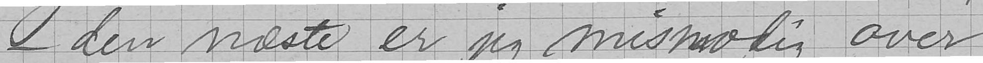- den næste er jeg mismodig over
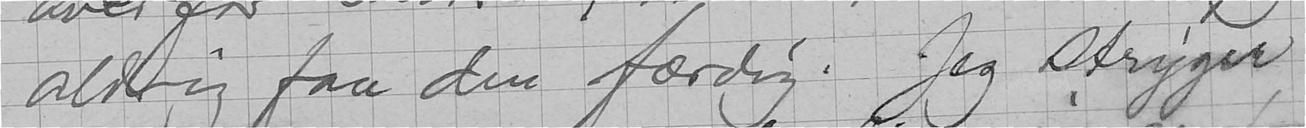aldrig faa den færdig. Jeg stryger
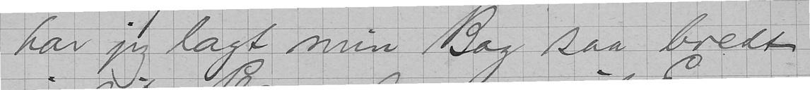har jeg lagt min Bog saa bredt
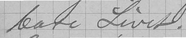bare Livet.
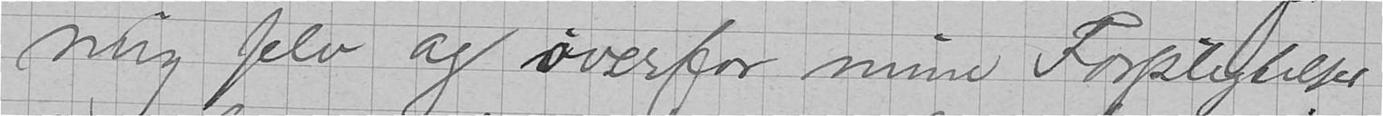mig selv og overfor mine Forpligtelser
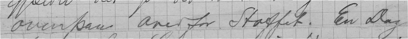ovenpaa overfor Stoffet. En Dag
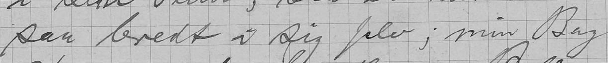saa bredt i sig selv; min Bog
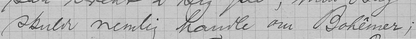skulde nemlig handle om Bohêmer;
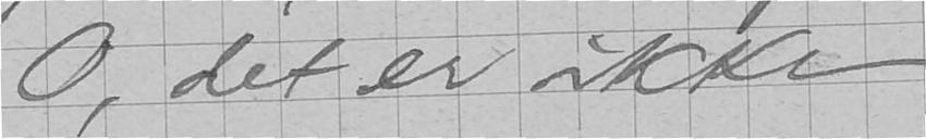O, det er ikke
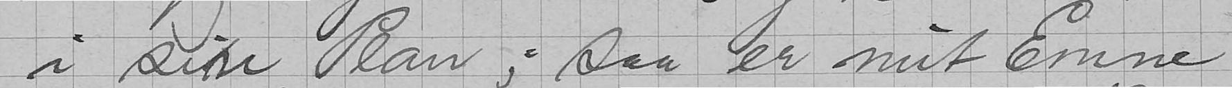i sin Plan; saa er mit Emne
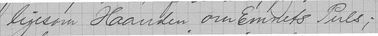ligesom Haanden om Emnets Puls;
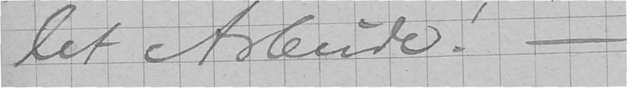let Arbeide! -
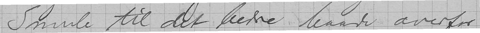Smule til det bedre baade overfor
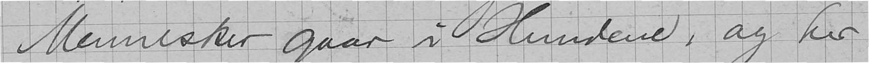Mennesker gaar i Hundene, og her
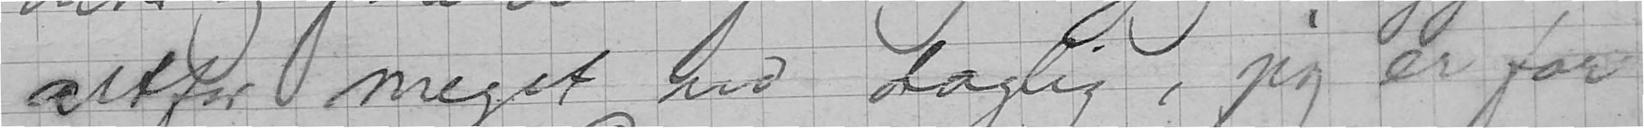altfor meget ud daglig, jeg er for
